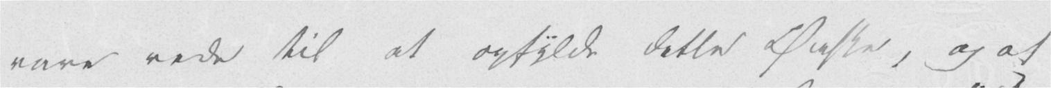vare rede til at opfylde dette Ønske, og at
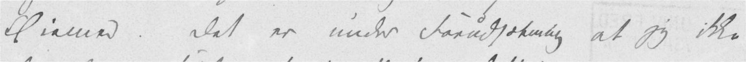Øiemed. Det er under Forudsætning at jeg ikke
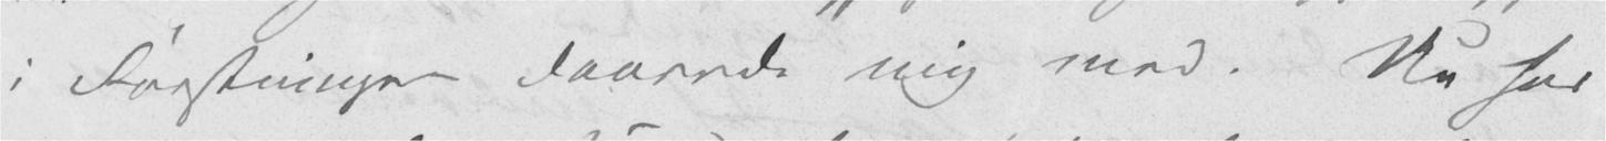i Førstningen daarede mig med. Nu har
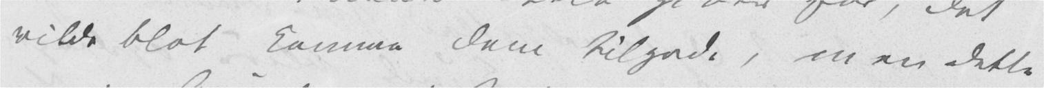vilde blot komme dem tilgode, men dette
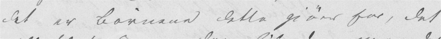det er Børnene dette gjøres for, det
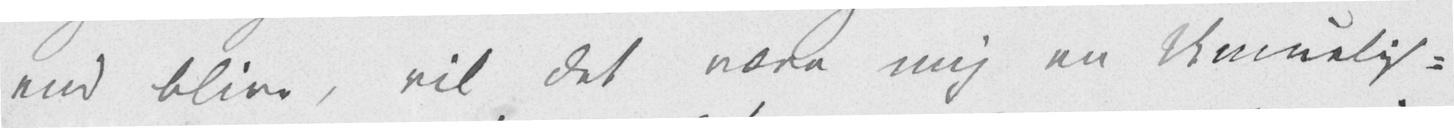end blive, vil det være mig en Umuelig-
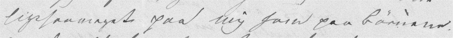ligesaameget paa mig som paa Børnene,
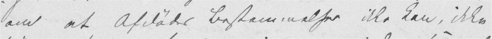om at Afdødes Bestemmelser ikke kan, ikke
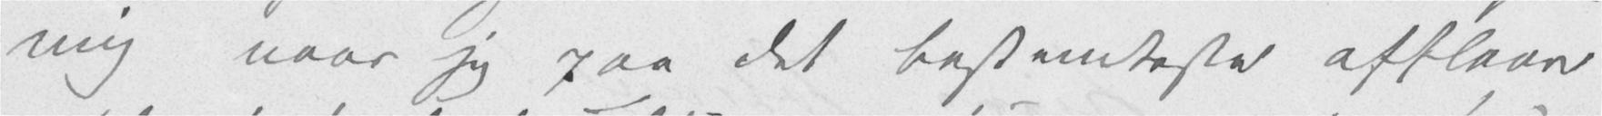mig naar jeg paa det bestemteste afslaaer
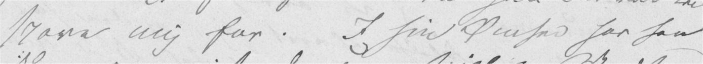spare mig for. I sin Ømhed har han
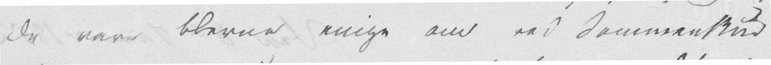de vare blevne enige om ved Sammenskud
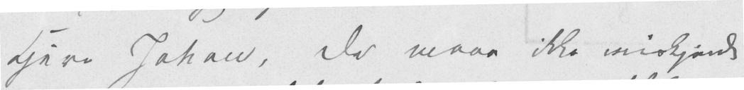Kjere Johan, De maae ikke miskjende
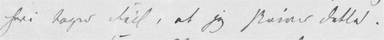heri tager Feil, at jeg skriver dette.
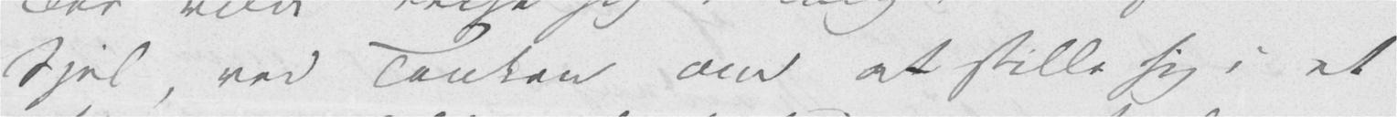Sjel, ved Tanken om at stille sig i et
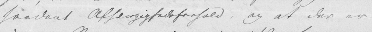saadant Afhængighedsforhold, og at der er
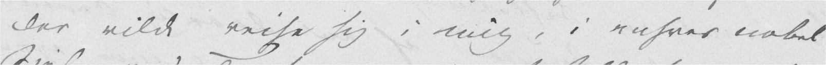der vilde reise sig i mig, i enhver nobel
